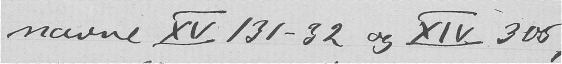navne XV 131-32 og XIV 305,
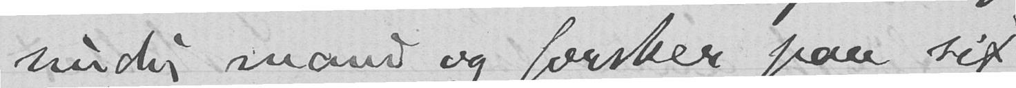sindig mand og forsker paa sit
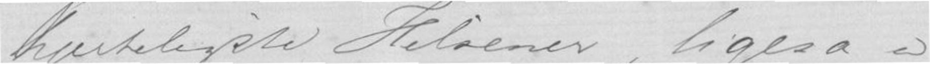hjerteligste Hilsener, ligesaa
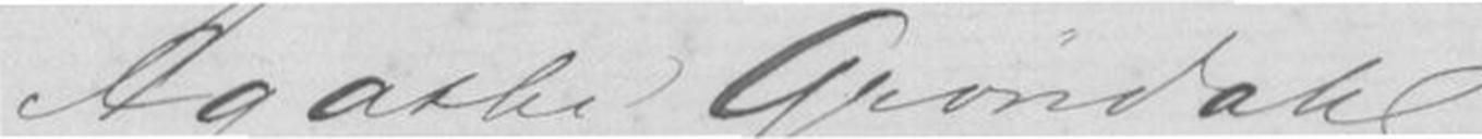Agathe Grøndahl
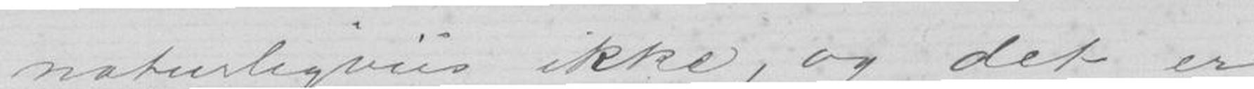naturligvis ikke, og det er
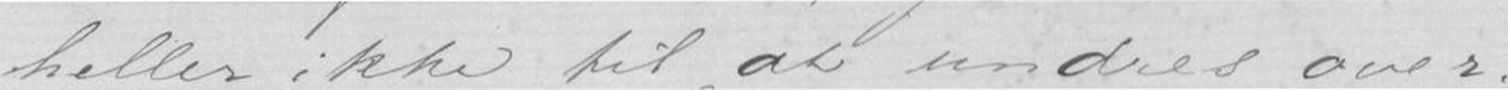heller ikke til at undres over;
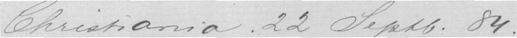Christiania. 22 Septb. 84.
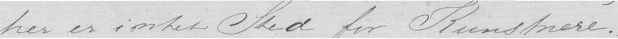her er intet Sted for Kunstnere.
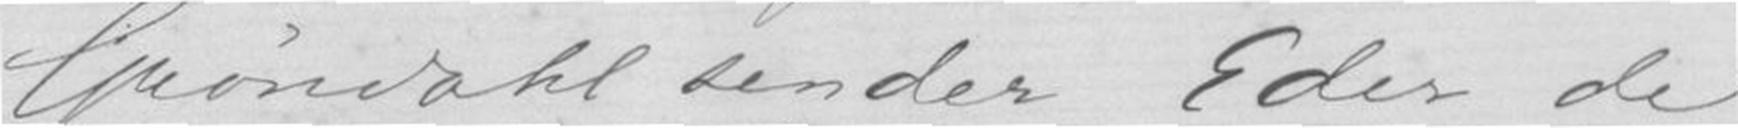Grøndahl sender Eder de
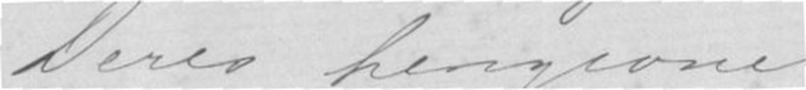Deres hengivne
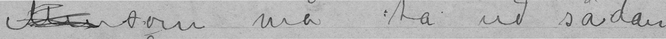Men som må ta ud sådan
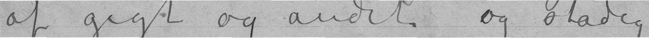af gigt og andet og stadig
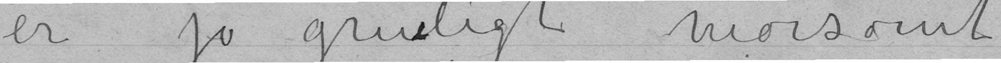er jo grueligt morsomt
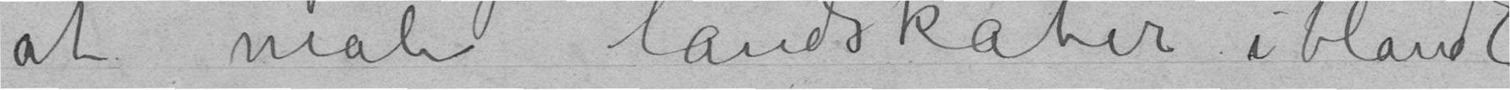at male landskaber iblandt
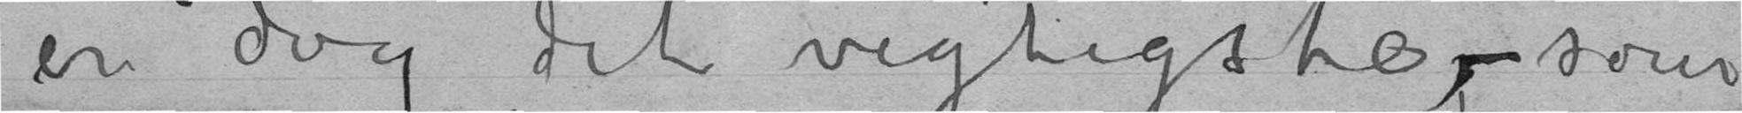er dog det vigtigste – som
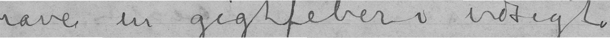have en gigtfeber i udsigt.
